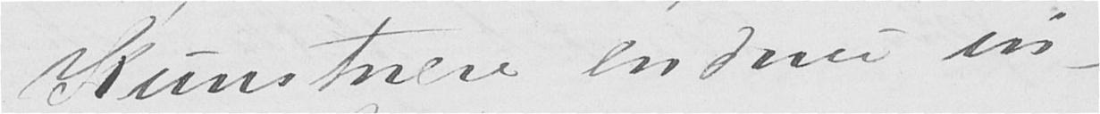Kunstnere endnu in-
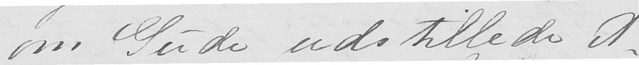om Gude udstillede A-
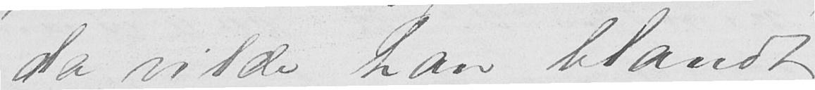da vilde han blandt
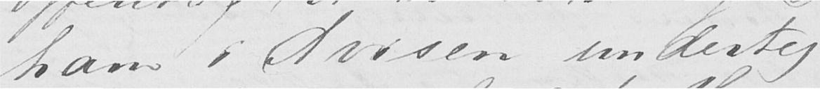ham i Avisen, underteg-
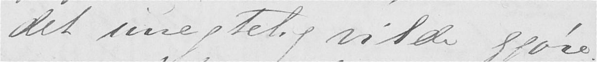det unegtelig vilde gjøre,
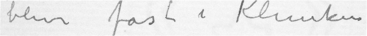blive fast i Klinikken
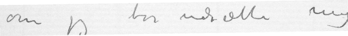om jeg bør udsætte mig
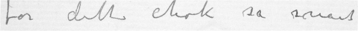for dette chok så snart
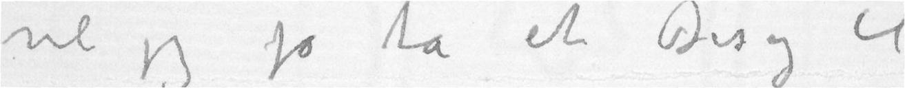vil jeg jo ta et Besøg til
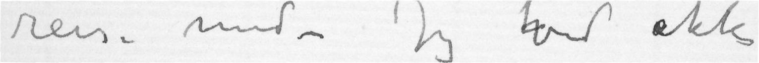reise med – Jeg ved ikke
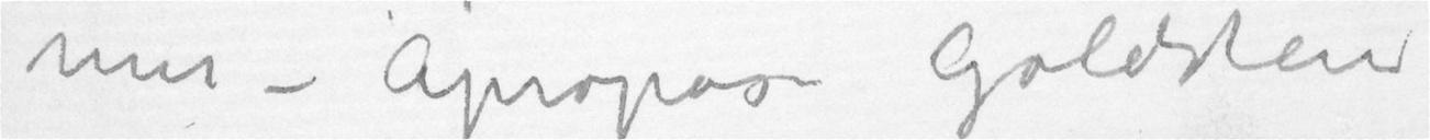mer – Apropos Goldstein
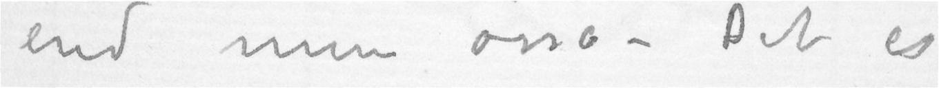end mine osså – Det er
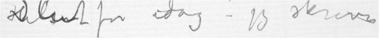Slut for idag – jeg skriver
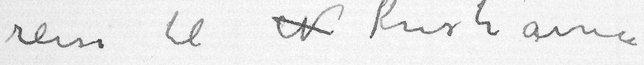reise til N Kristiania
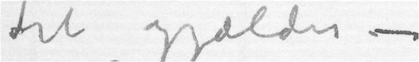det gjælder –
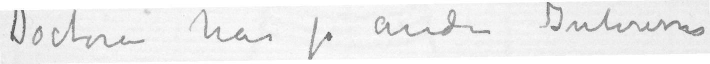Doctoren har jo andre Interesser
